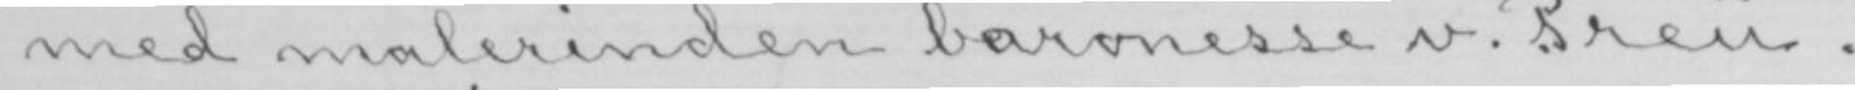med malerinden baronesse v. Preu-
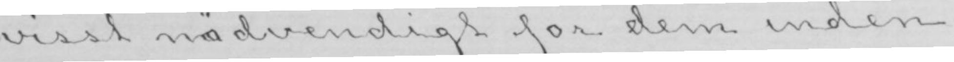visst nødvendigt for dem inden
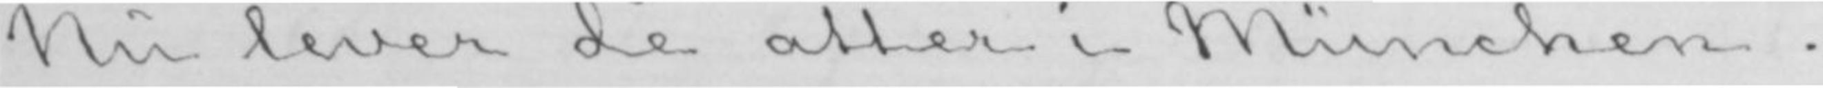Nu lever de atter i München.
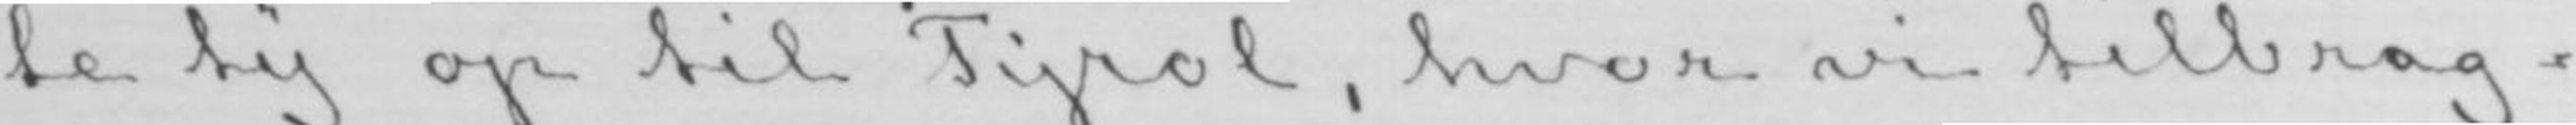te ty op til Tyrol, hvor vi tilbrag-
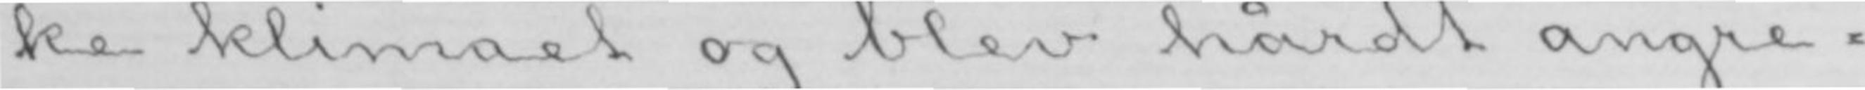ke klimaet og blev hårdt angre-
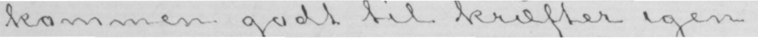kommen godt til kræfter igen.
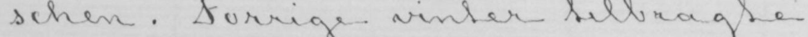schen. Forrige vinter tilbragte
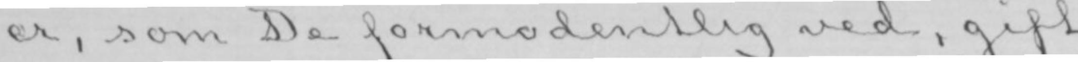er, som De formodentlig ved, gift
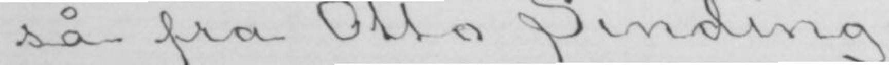så fra Otto Sinding.
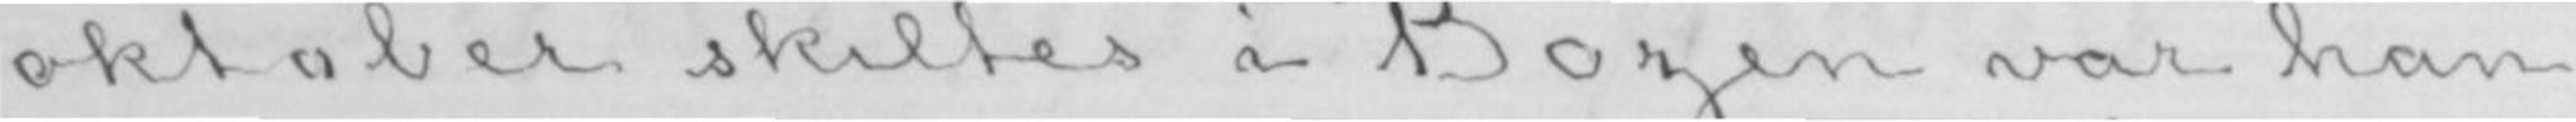oktober skiltes i Bozen var han
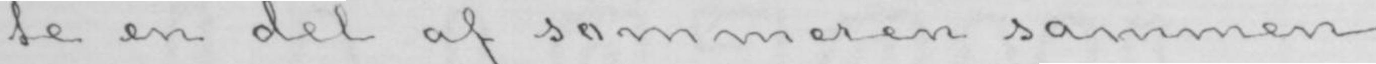te en del af sommeren sammen
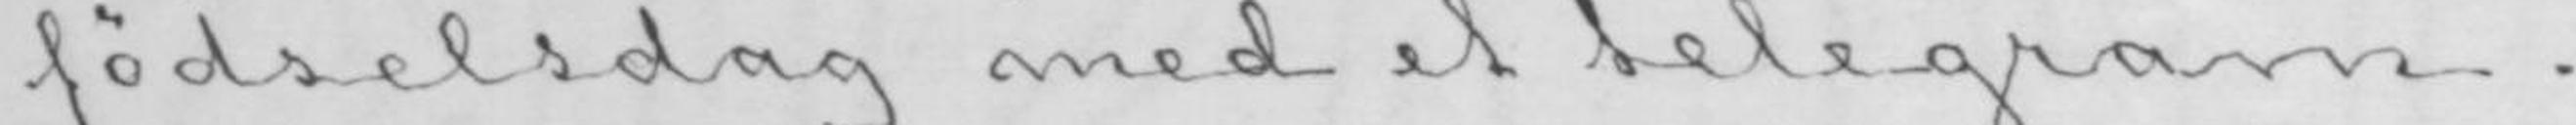fødselsdag med et telegram.
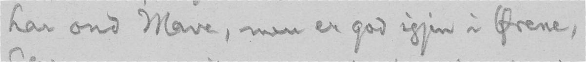har ond Mave, men er god igjen i Ørene,
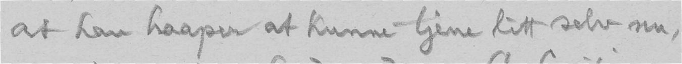at han haaper at kunne tjene litt selv nu,
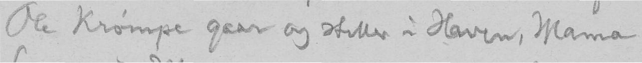Ole Krømpe gaar og steller i Haven, Mama
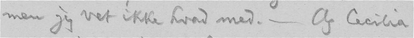men jeg vet ikke hvad med. - Og Cecilia
